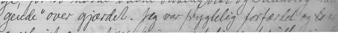gende" over gjærdet. Jeg var frygtelig forfærdet og l er
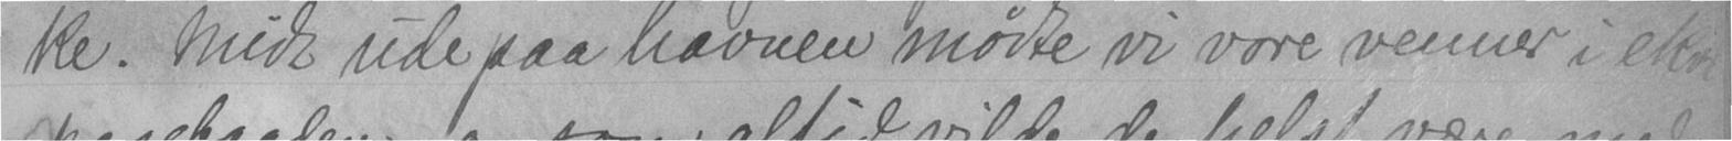ke. Midt ude paa havnen mødte vi vore venner i ekvi-
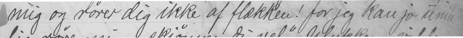mig og rører dig ikke af flækken! for jeg kan jo umu-
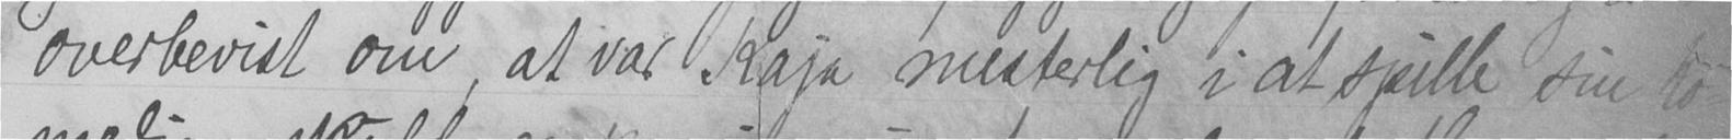overbevist om, at var Kaja mesterlig i at spille sin ko-
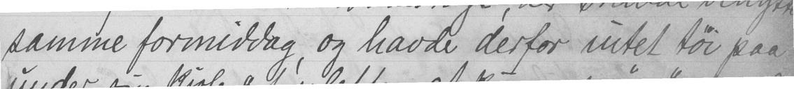samme formiddag, og havde derfor intet tøi paa
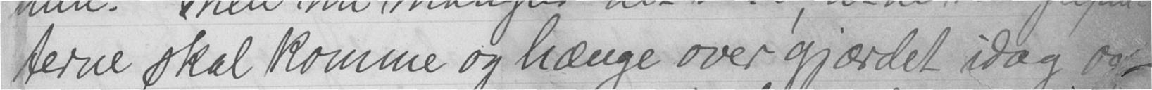terne skal komme og hænge over gjærdet idag og-
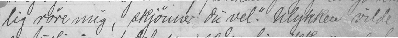lig røre mig, skjønner du vel." Ulykken vilde
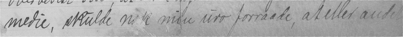medie, skulde nok min uro forraade, at eller andet
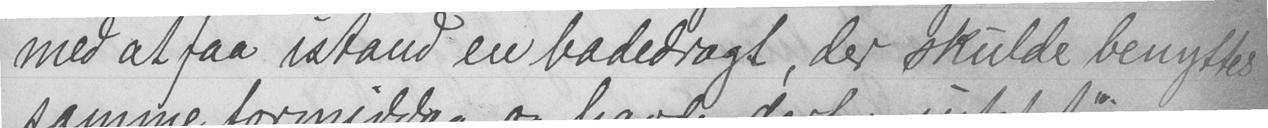med at faa istand en badedragt, der skulde benyttes
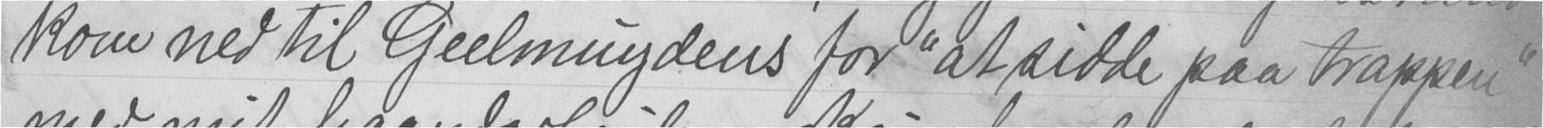kom ned til Geelmuydens for "at sidde paa trappen"
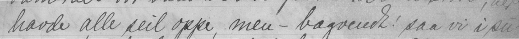havde alle seil oppe, men - bagvendt! saa vi i su-
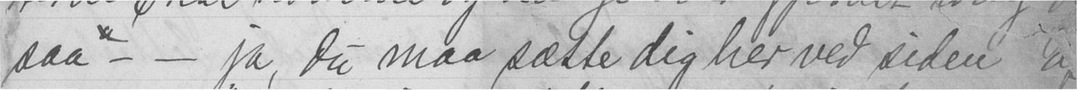saa" - - ja, du maa sætte dig her ved siden af
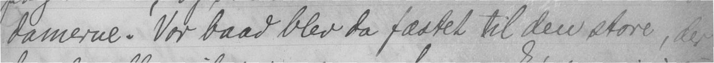damerne. Vor baad blev da fæstet til den store, der
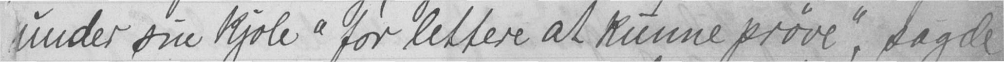under sin kjole "for lettere at kunne prøve", sagde
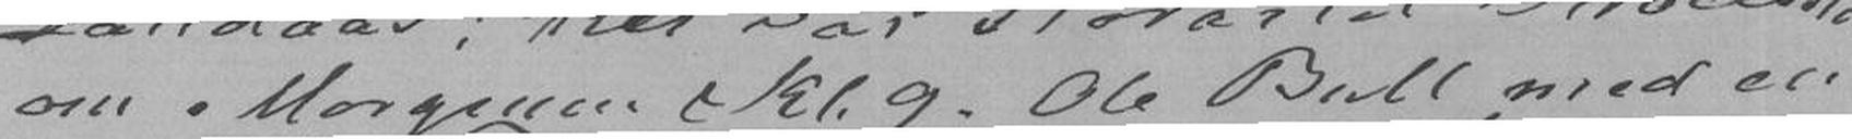om Morgenen Kl.9. Ole Bull med en
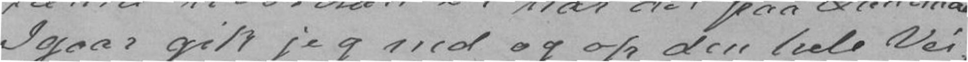Igaar gik jeg ned og op den hele Vei,
